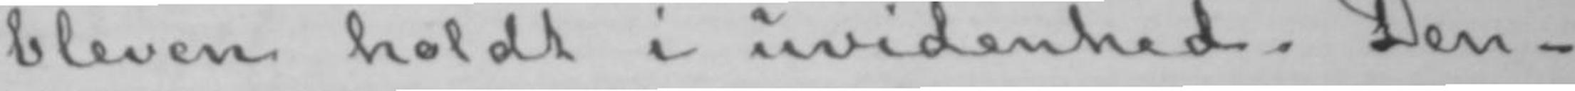bleven holdt i uvidenhed. Den-
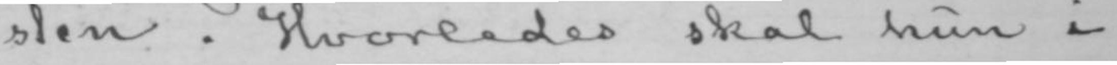sten. Hvorledes skal hun i
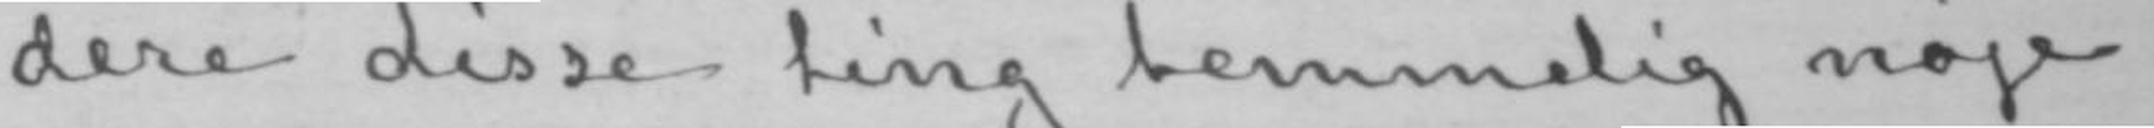dere disse ting temmelig nøje,
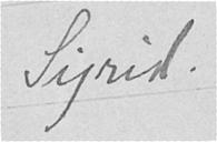Sigrid.
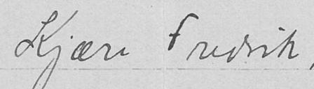Kjære Fredrik,
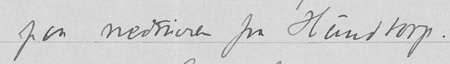paa nedturen fra Hundtorp.
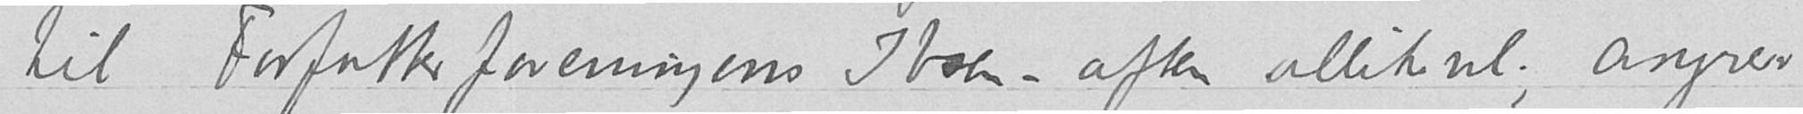til Forfatterforeningens Ibsen-aften allikevel; angrer
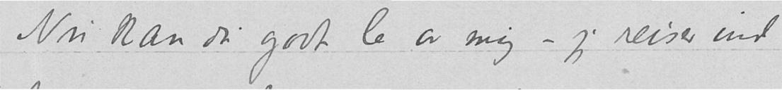Nu kan du godt le av mig – jeg reiser ind
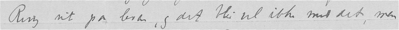Ring ut paa leven, og det blir vel ikke med det, men
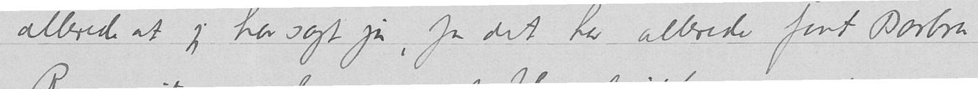allerede at jeg har sagt ja, for det har allerede faat Barbra
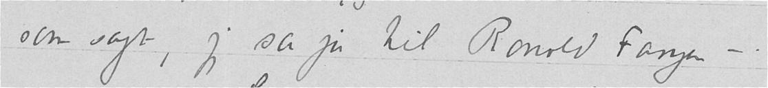som sagt, jeg sa ja til Ronald Fangen -.
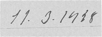11.3.1928
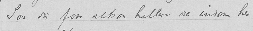Saa du faar altsaa hellere se indom her
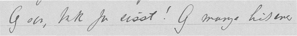Og saa, tak for sisst! Og mange hilsener
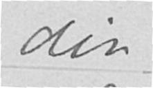din
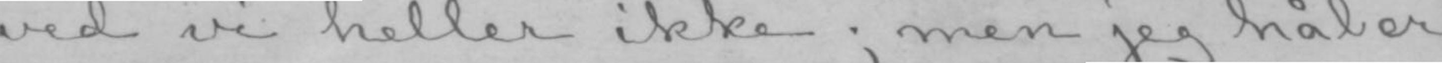ved vi heller ikke; men jeg håber
